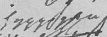hyppigen
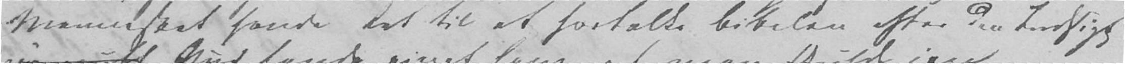Mennesket havde Ret til at fortolke Bibelen efter den Indsigt
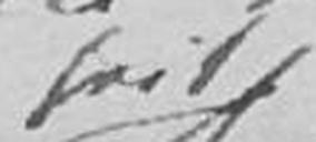frit
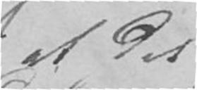at det
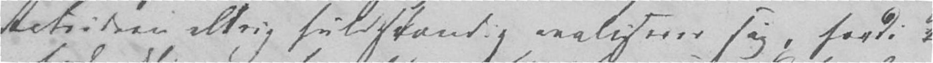Retsideen aldrig fuldstændig realiserer sig, fordi
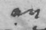en
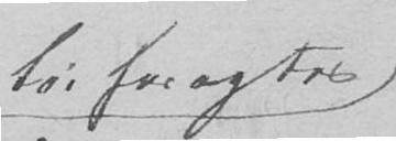bør foragtes
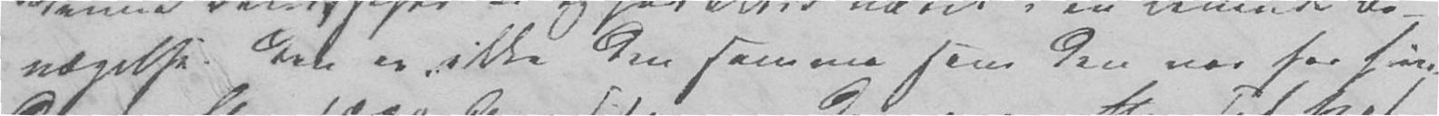vægelse. Den er ikke den samme som den var for hun-
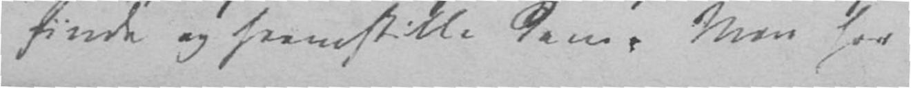finde og freemstille dem. Man har
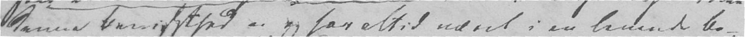denne Bevidsthed er og har altid været i en levende Be-
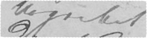Begrebet
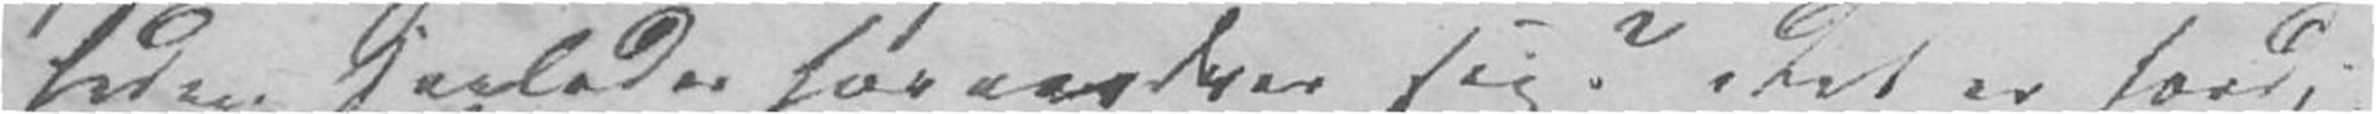heden saaledes forandrer sig? Det er fordi
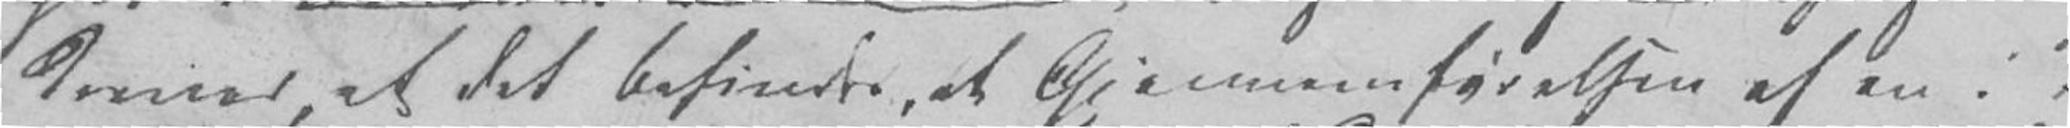derved, at det befindes, et Gjennemførelsen af en i
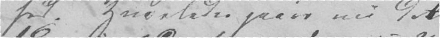het. Hvorledes gaaer nu det
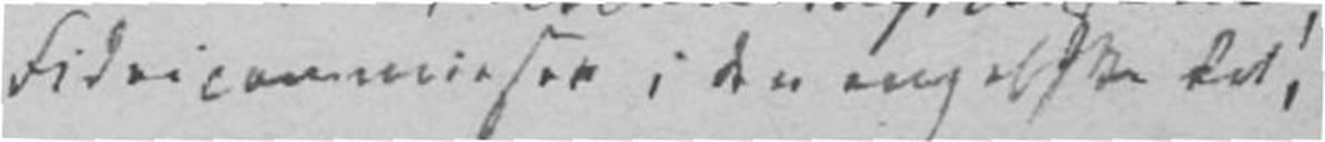Fideicommisser i den engelske Ret,
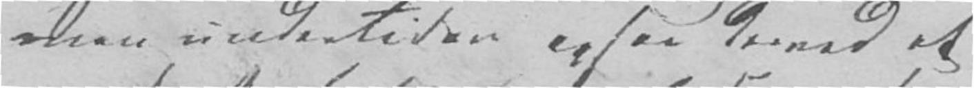men undertiden ogsaa derved at
